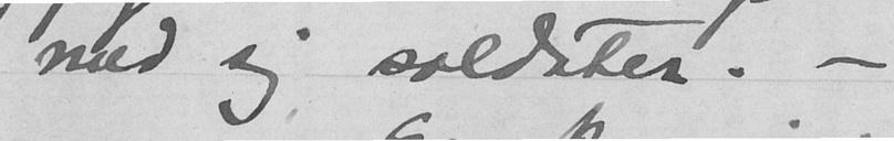med sig soldater. -
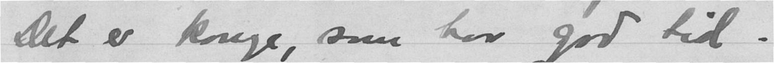Det er konge, som har god tid.
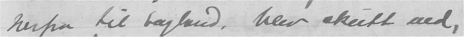herfra til England, blev skutt ned,
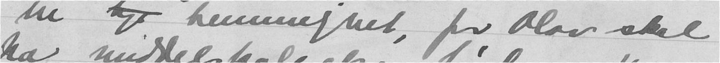en bys hemmelighet, for Olav skal
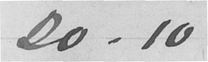20-10
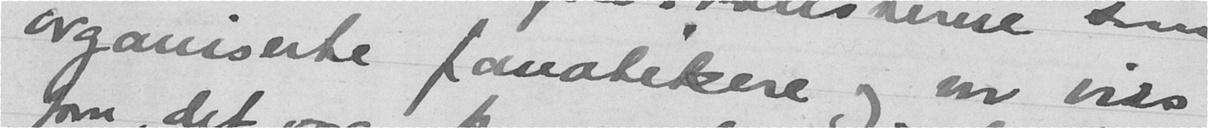organiserte fanatikere og var viss
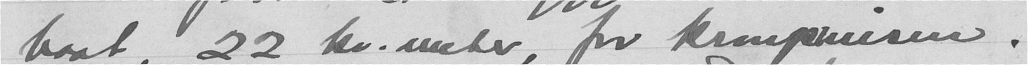baat, 22 kv. meter, for kronprinsen.
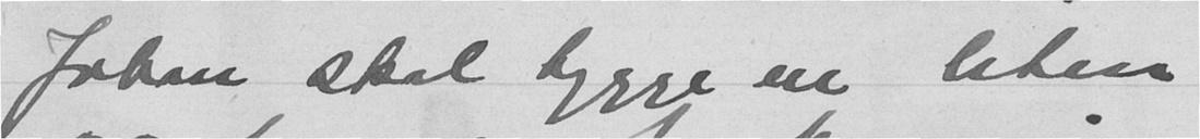Johan skal bygge en liten
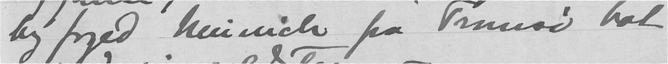byfoged Meinich fra Tromsø hat
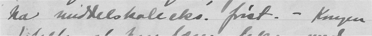ha middelskoleeks. først. - Kongen
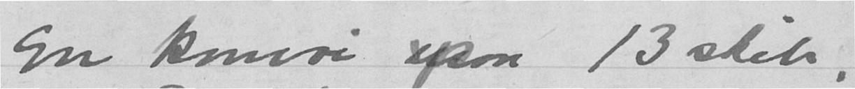En konvoi paa 13 skib,
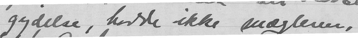gydelse, hadde ikke mægleren,
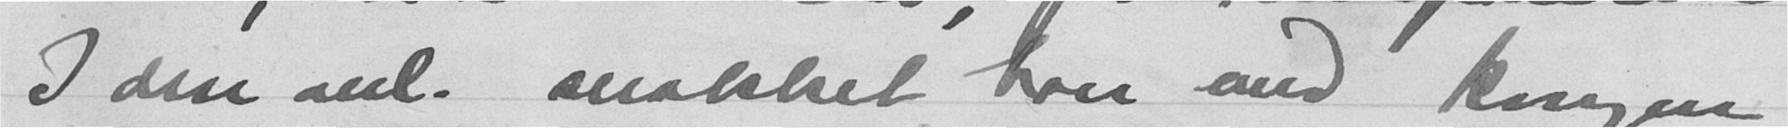I den anl. snakket han med kongen
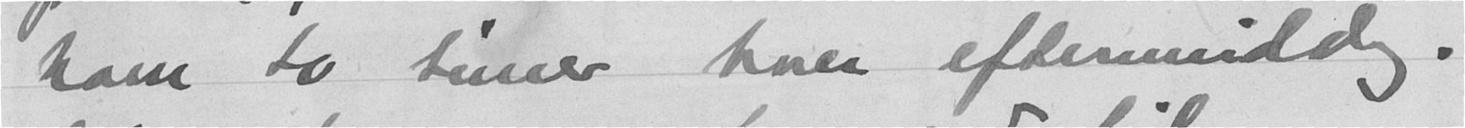ham to timer hver eftermiddag.
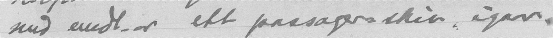med emdt.er ett passagersskib, igaar.
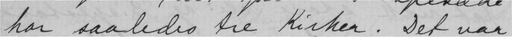har saaledes tre Kirker. Det var
